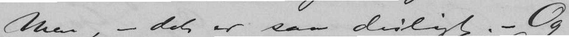Men, - det er saa deiligt. - Og
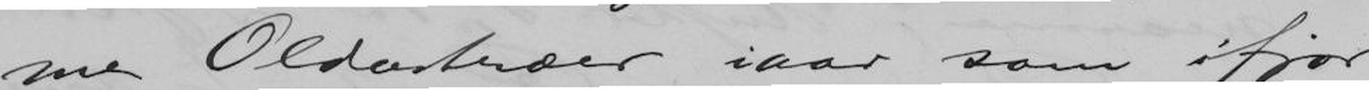me Oldertræer iaar som ifjor
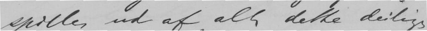spille ud af alt dette deilige
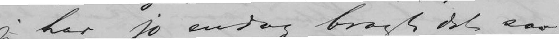jeg har jo endog bragt det saa-
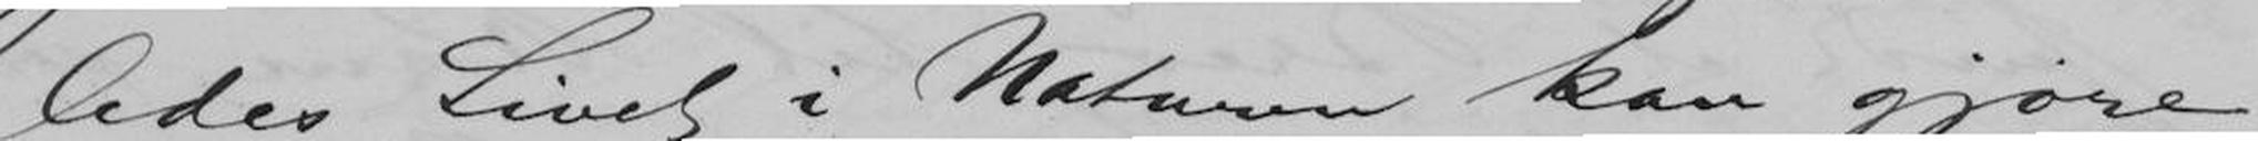ledes Livet i Naturen kan gjøre
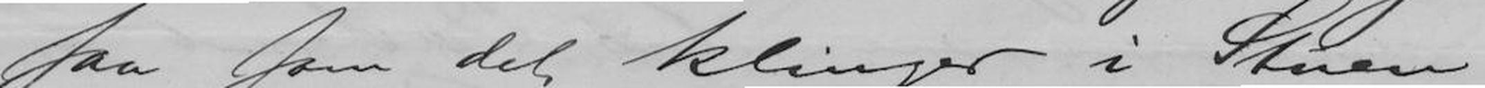saa som det klinger i Stuen
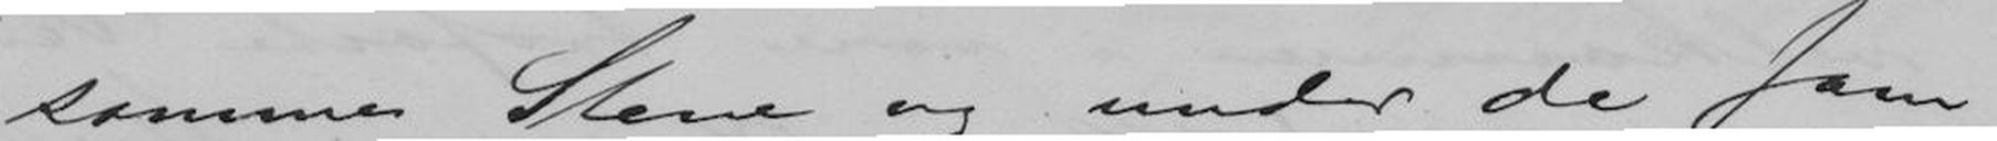samme Stene og under de sam-
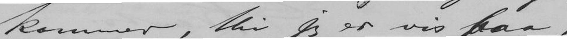kommer, thi jeg er vis paa,
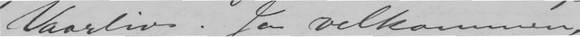Vaarliv. Ja velkommen,
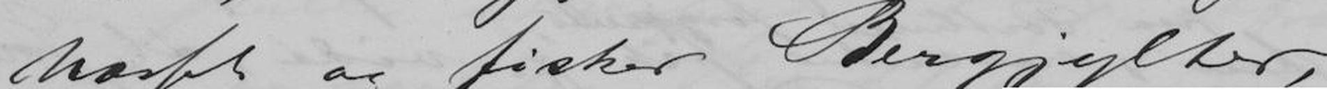Næsset og fisker Bergjylter,
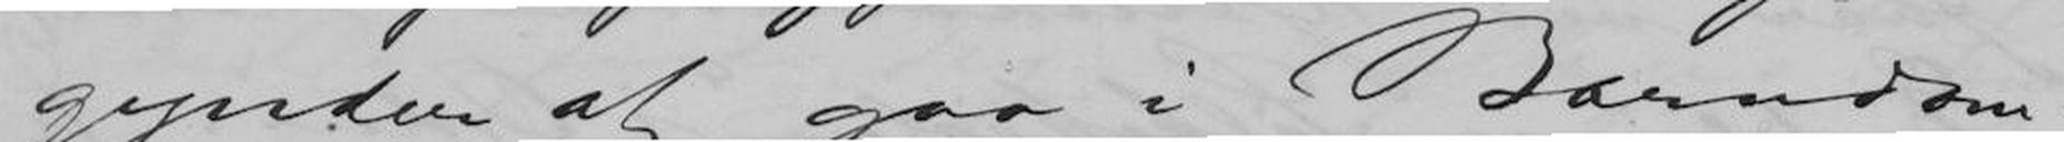gynder at gaa i Barndom-
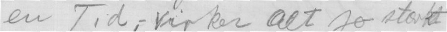en Tid, virker Alt jo stærkt
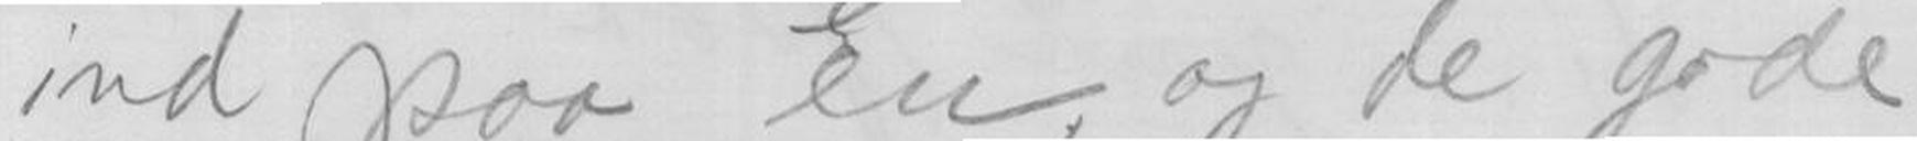ind paa En, og de gode
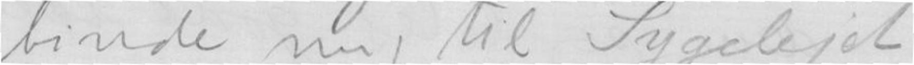binde mig til Sygelejet
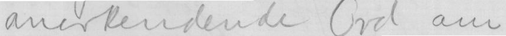anerkendende Ord om
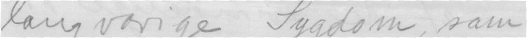langvarige Sygdom, som
